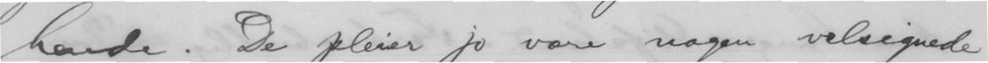hende. De pleier jo være nogen velsignede
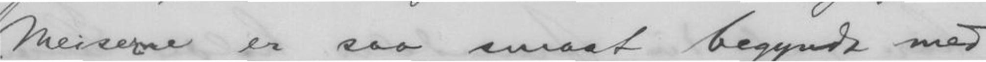Meiserne er saa smaat begyndt med
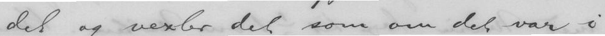det og vexler det som om det var i
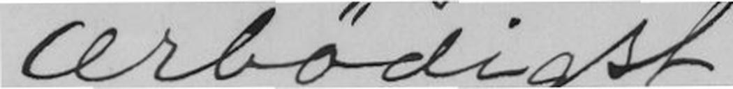ærbødigst
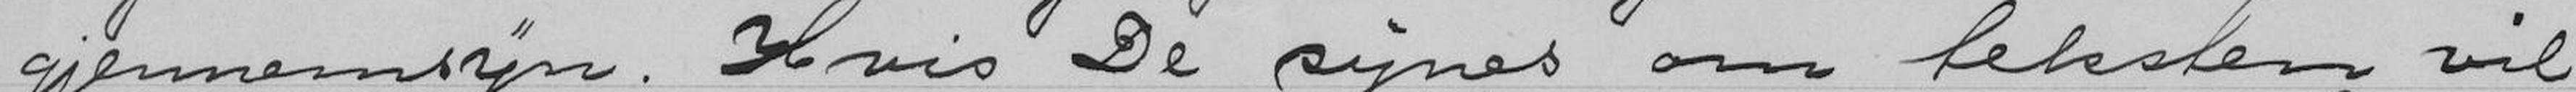gjennemsyn. Hvis De synes om teksten vil
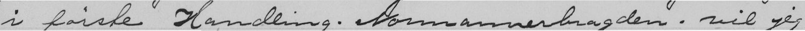i første Handling. Normabragden vil jeg
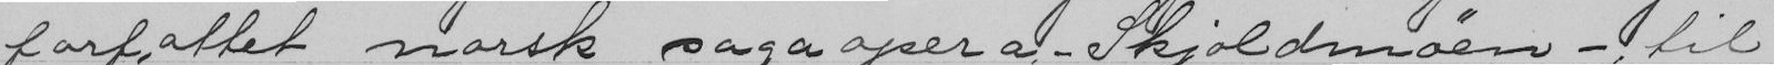forfattet norsk sagaopera - Skjoldmøen - til
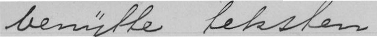benytte teksten
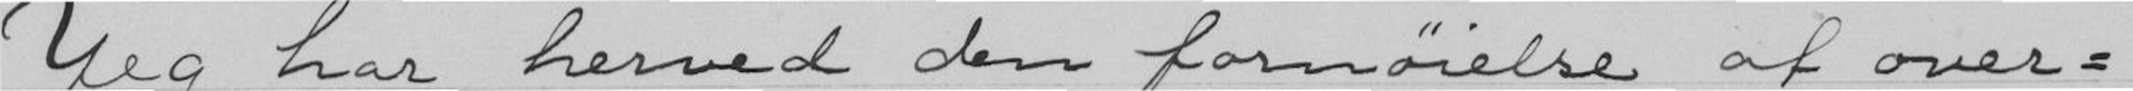Jeg har herved den fornöielse at over=
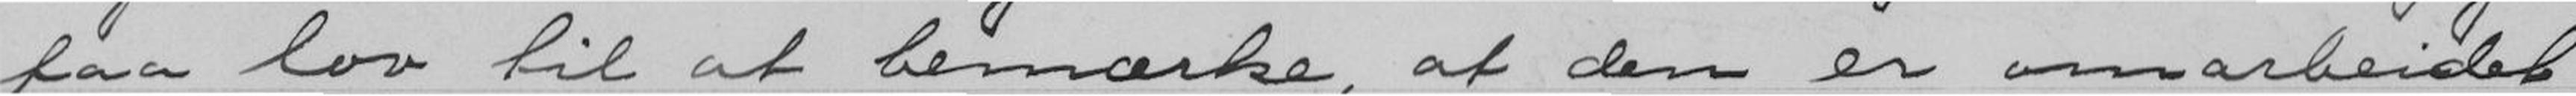faa lov til at bemærke, at den er omarbeidet
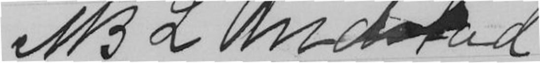MB L Amd
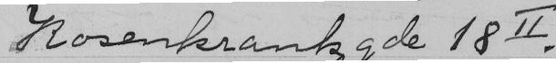Rosenkrantzgde 18 II
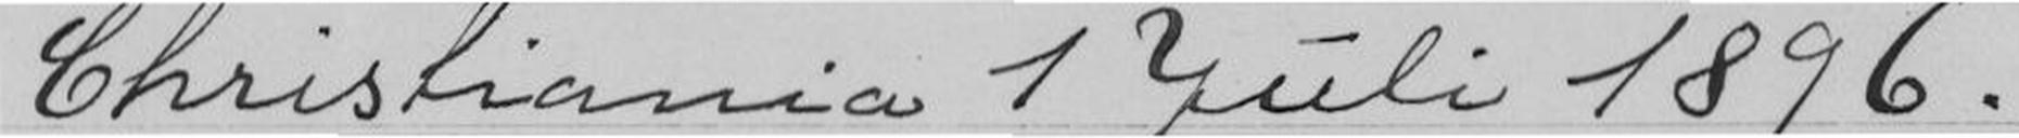Christiania 1 Juli 1896
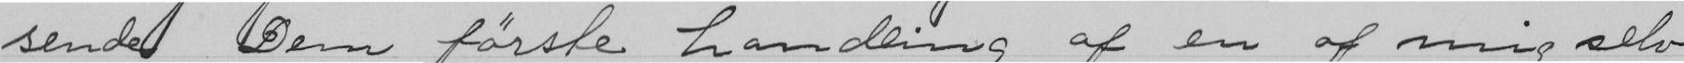sende Dem første handling af en af mig selv
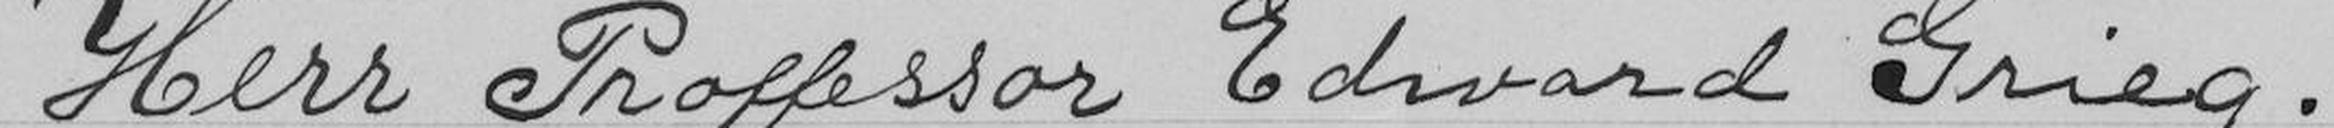Herr Proffessor Edward Grieg.
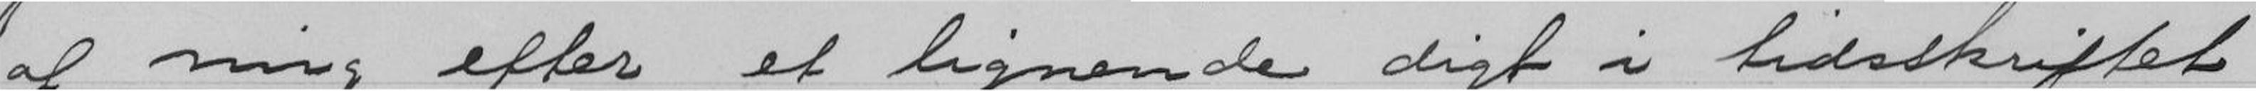af mig efter et lignende digt i tidsskriftet
